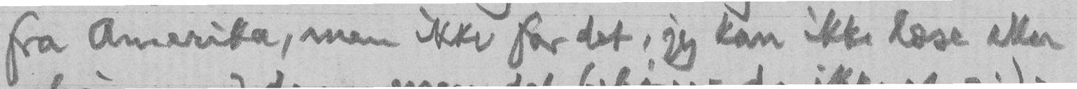fra Amerika, men ikke for det, jeg kan ikke læse eller
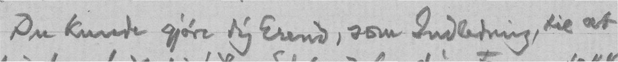Du kunde gjøre dig Erend, som Indledning, til at
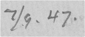7/9. 47.
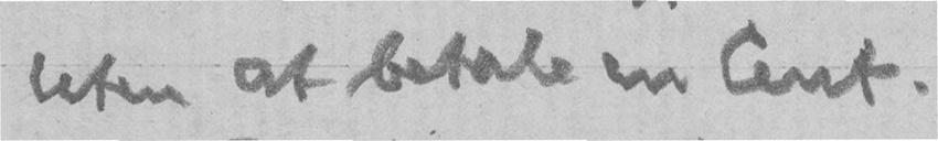uten at betale en Cent.
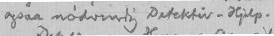ogsaa nødvendig Detektiv-Hjelp.
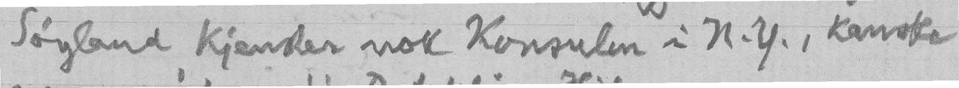Søyland kjender nok Konsulen i N.Y., kanske
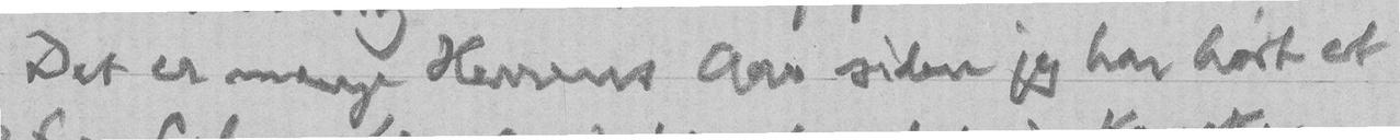Det er mange Herrens Aar siden jeg har hørt et
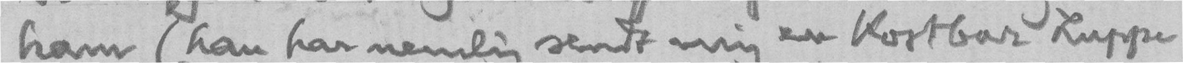ham (han har nemlig sendt mig en kostbar Luppe
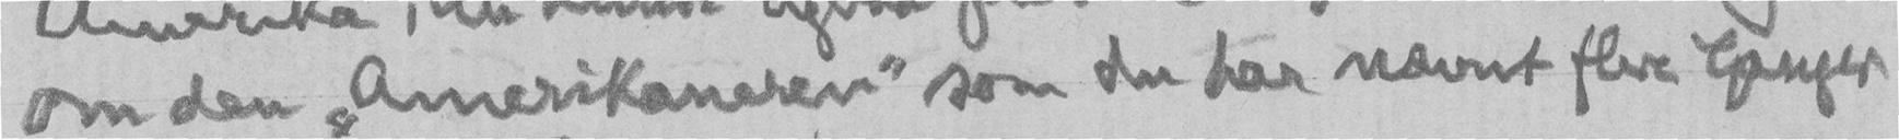om den "Amerikaneren" som du har nævnt flere Ganger
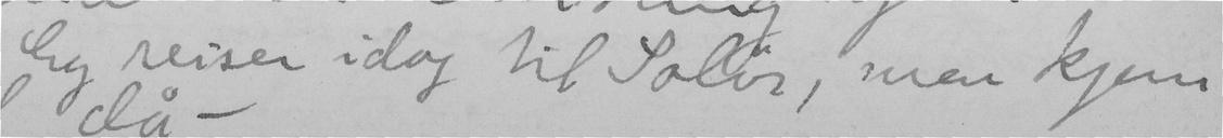Eg reiser idag til Solör, men kjem
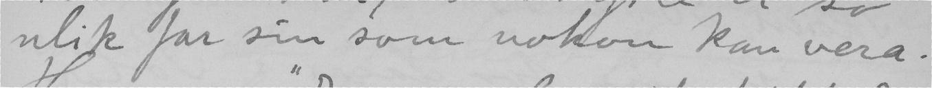ulik far sin som nokon kan vera.
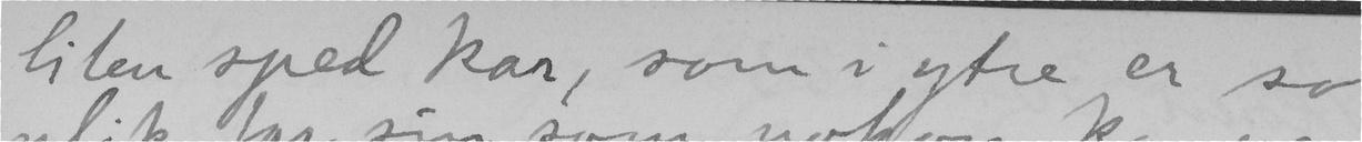liten sped kar, som i ytre er so
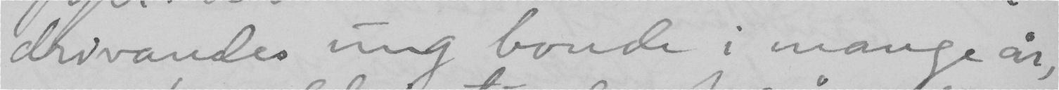drivandes ung bonde i mange år,
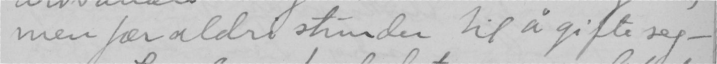men fær aldri stunden til å gifte seg –
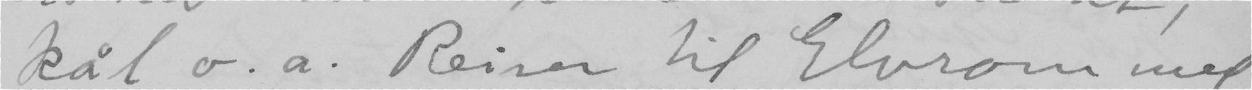kål o.a. Reiser til Elvrom med
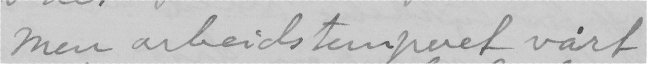Men arbeidstempoet vårt
